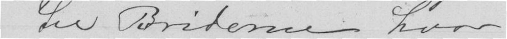og til Briderne hvor
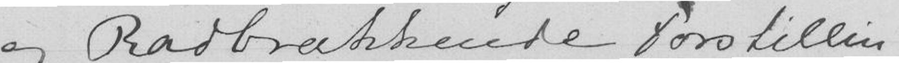og Radbrækkende Forestillin-
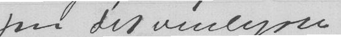paa det venligste.
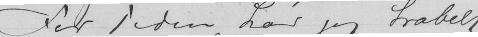For Tiden har jeg trabelt
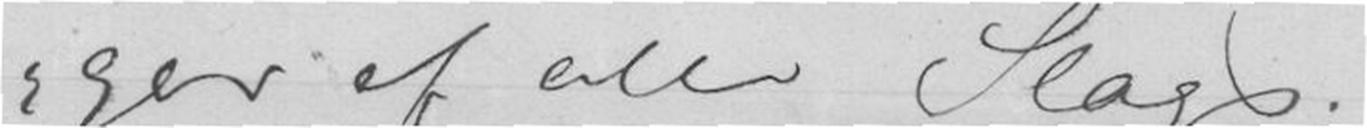ger af alle Slags.
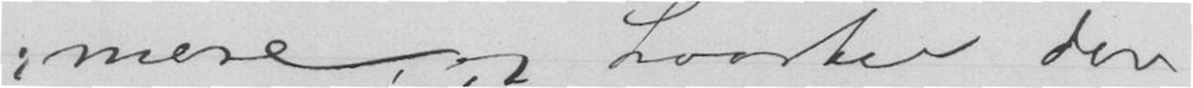merem og hvortil den
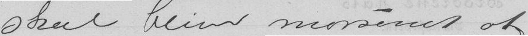skal blive morsomt at
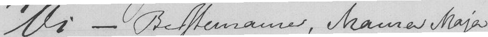Vi - Bedstemama, Mama Maja
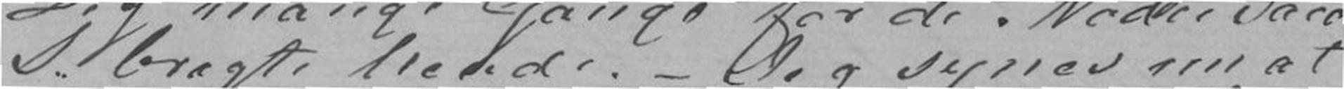S. bragte hende. - Jeg synes nu at
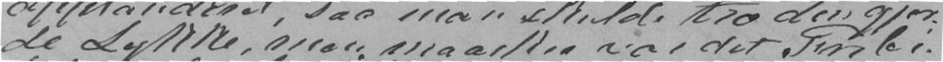de Lykke, men maaske var det Fribi-
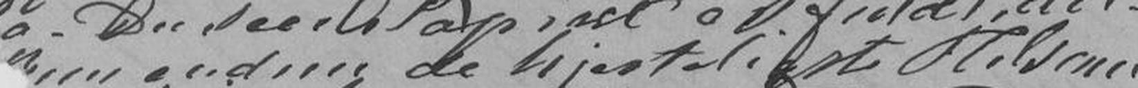endnu de hjerteligste Hilsener
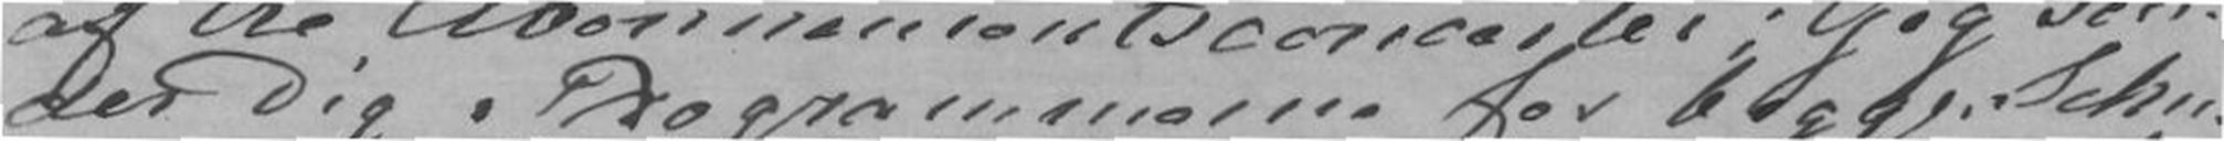der Dig Programmerne for begge. Schu-
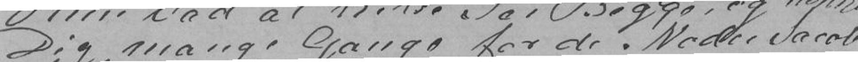Dig mange Gange for de Noder Jacob
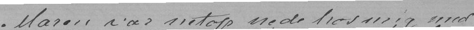Maren var netop nede hos mig med
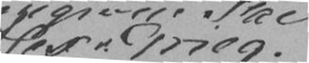Alexr. Grieg.
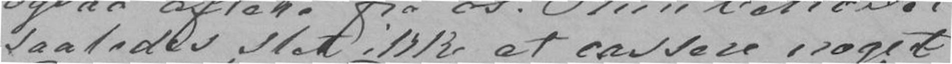saaledes slet ikke at cassere noget
